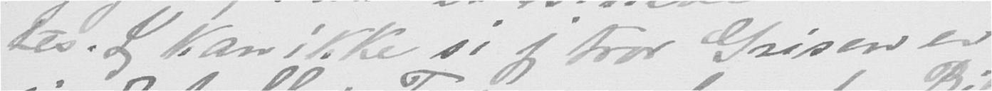tes. Jeg kan ikke si jeg tror Grisen er
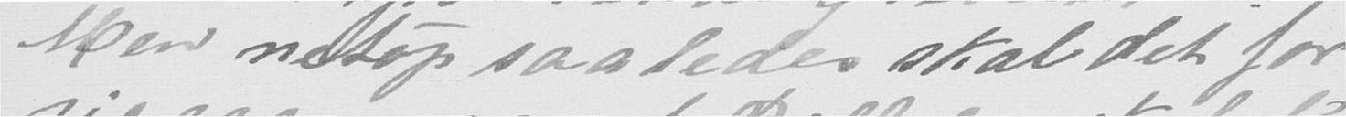Men netop saaledes skal det for
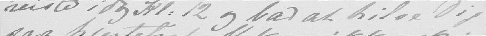reiste i dag Kl: 12 og bad at hilse Dig
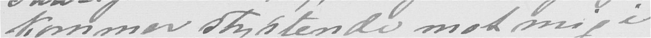kommer styrtende mot mig i
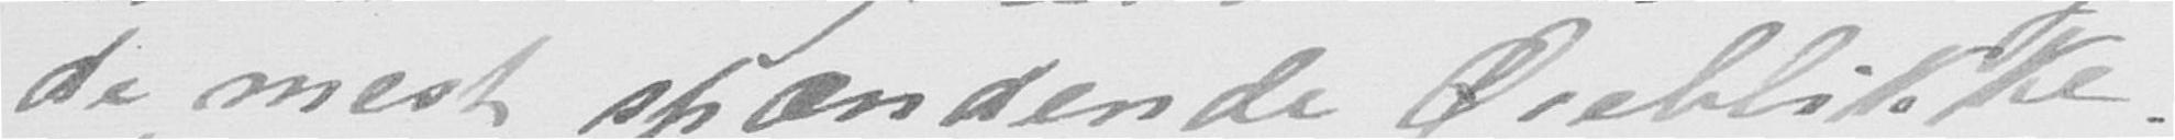de mest spændende Øieblikke.
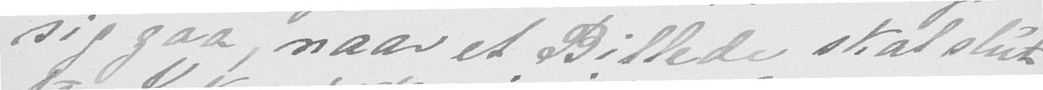sig gaa, naar et Billede skal slut-
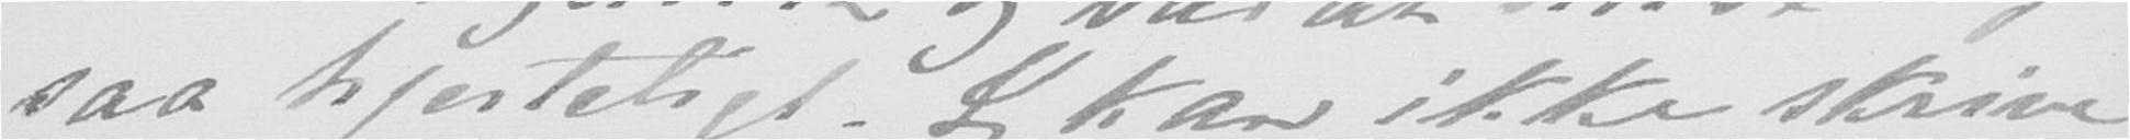saa hjerteligt. Jeg kan ikke skrive
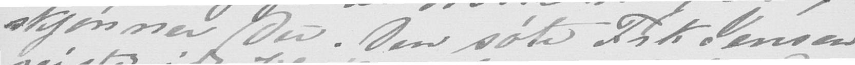skjønner Du. Den søte Frk Jensen
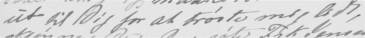ut til Dig for at trøste mig lidt,
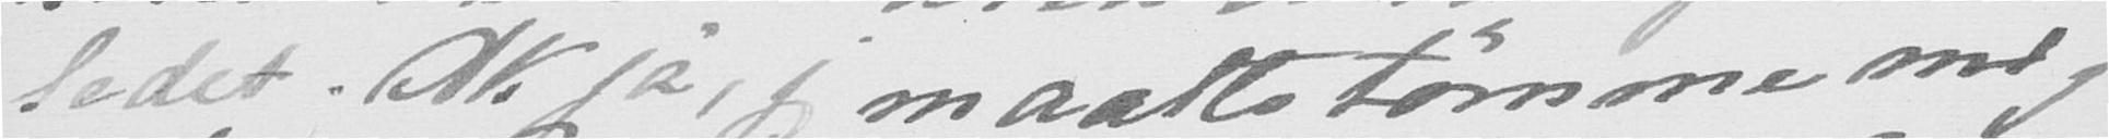ledet. Ak ja, jeg maatte tømme mig
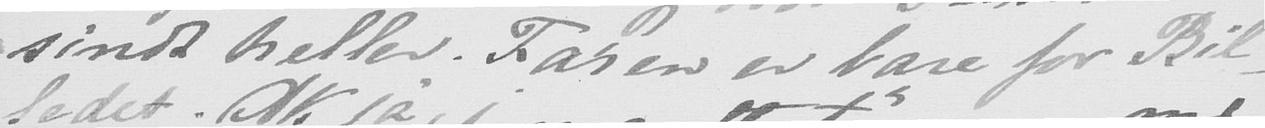sindt heller. Faren er bare for Bil-
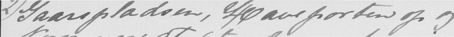Gaarspladsen, Haveporten op og
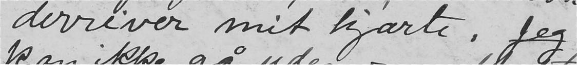derever mit hjærte, jeg
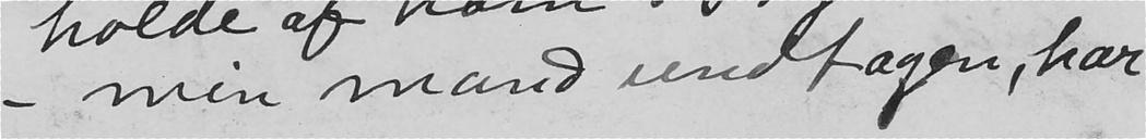- min mand undtagen, har
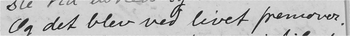Og det blev ved livet fremover.
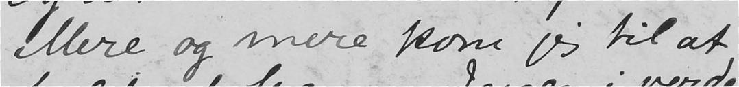Mere og mere kom jeg til at
